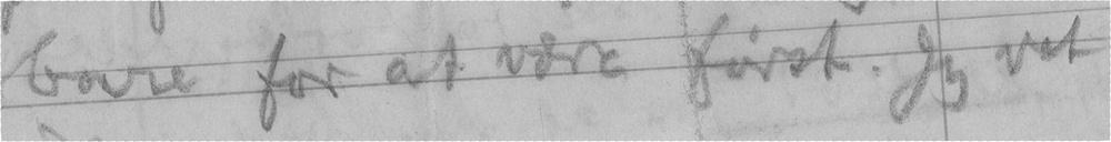bare for at være først. Jeg vet
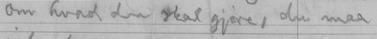om hvad du skal gjøre, du maa
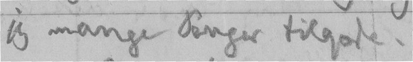jeg mange Penger tilgode.
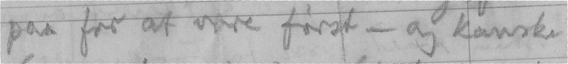paa for at være først - og kanske
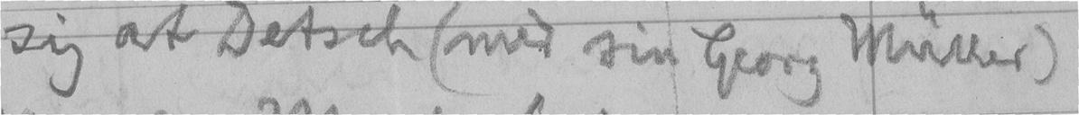sig at Detsch (med sin Georg Müller)
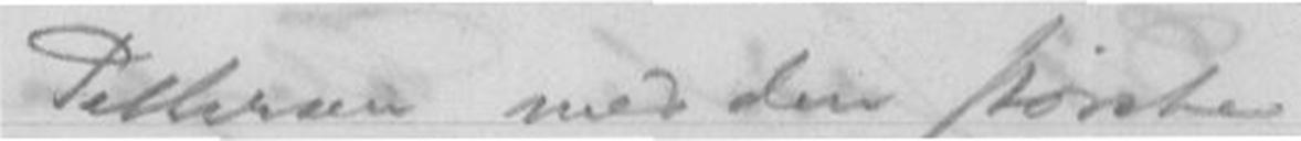Pettersen med den største
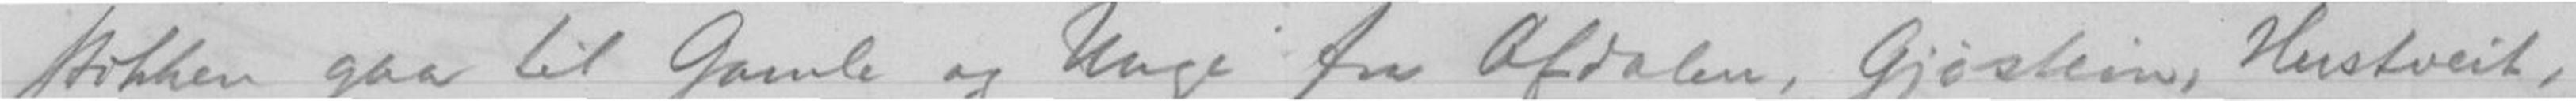stikken gaa til Gamle og Unge fra Afdalen, Gjøstein, Hustveit,
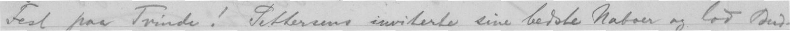Fest paa Tvinde! Pettersens inviterte sine bedste Naboer og lod Bud-
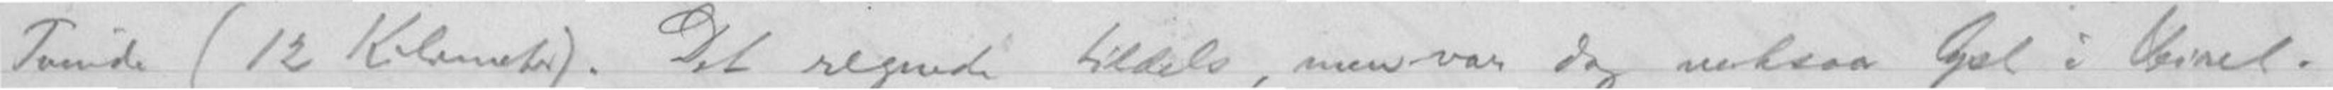Tvinde (12 Kilometer). Det regnede tildels, men var dog noksaa G... i Veiret.
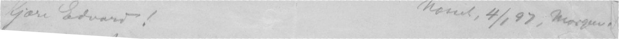Næsset, 4/1 97, Morgen Kjære Edvard!
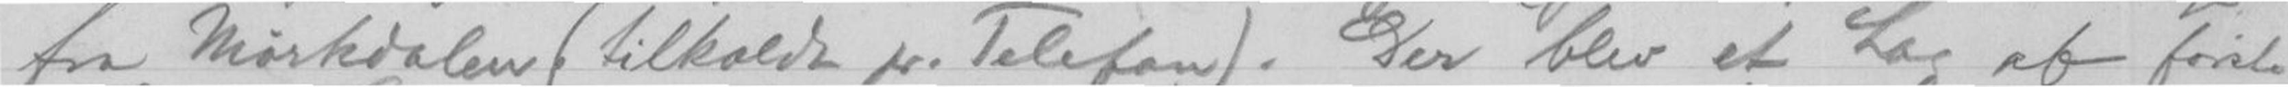fra Mørkdalen (tilkaldt pr. Telefon). Der blev et Lag af første
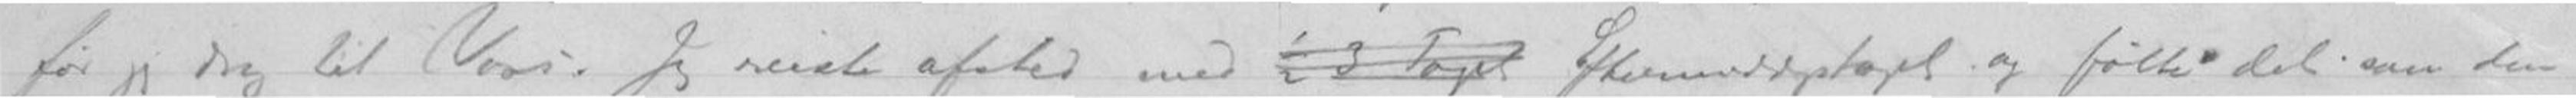før jeg drog til Voss. Jeg reiste afsted med ½ 3 Toget Eftermiddagstoget og følte det som den
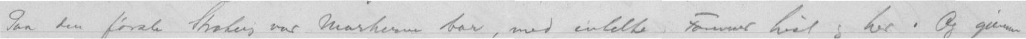Paa den første Strækning var Markerne bar, med enkelte Fonner hist og her. Og gjennem
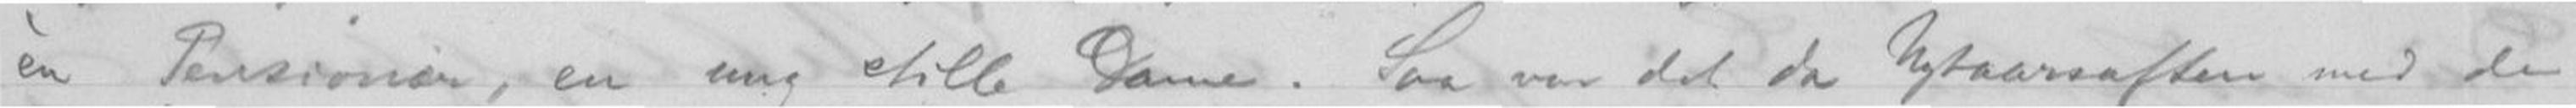èn Pensionær, en ung stille Dame. Saa var det da Nytaarsaften med de
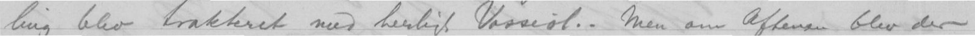ling blev trakteret med herligt Vosseøl. Men om Aftenen blev der
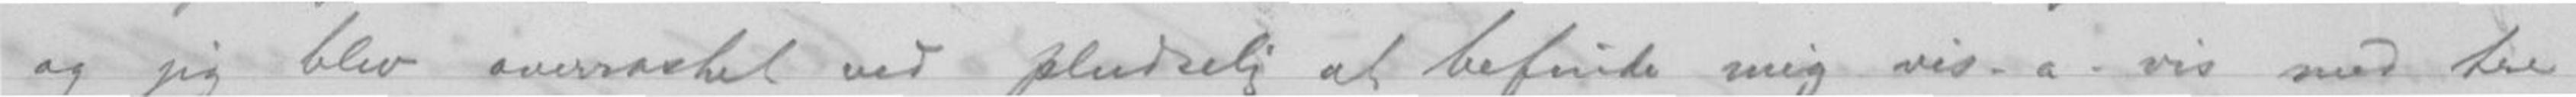og jeg blev overrasket ved pludselig at befinde mig vis-a-vis med tre
